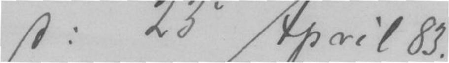D: 23 April 83.
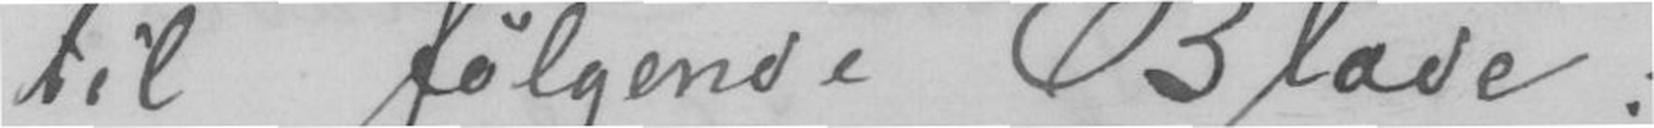til følgende Blade:
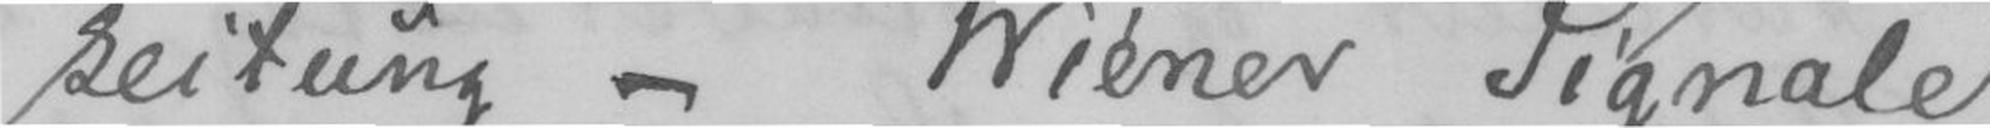reiting - Wièner Signale
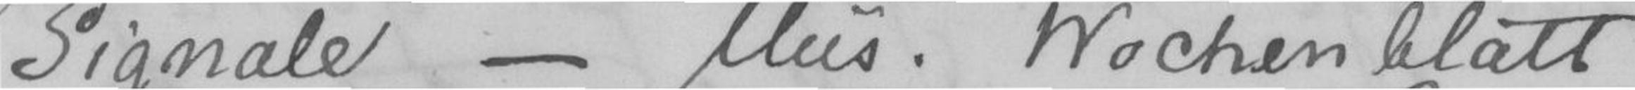Signale - Mus. Wochenblatt
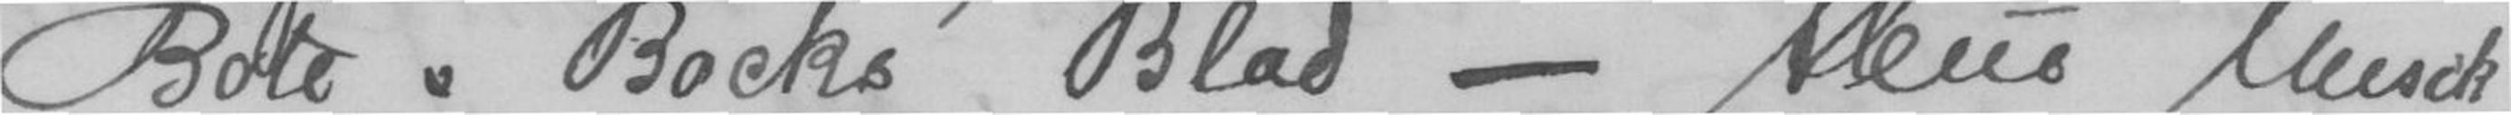Bote Bocks Blad- Neus Musik
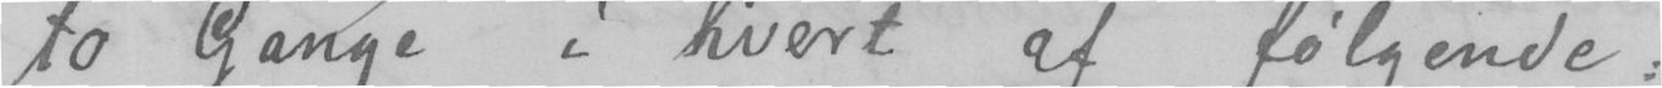to Ganger i hvert af følgende:
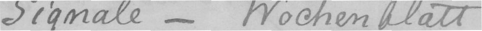Signale - Wochenblatt
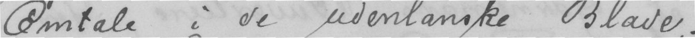Omtale i de udenlanske Blade
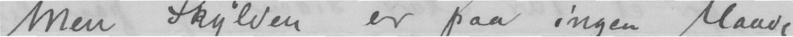Men Skylden er paa ingen Maade
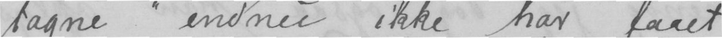tagne" endnu ikke har faaet
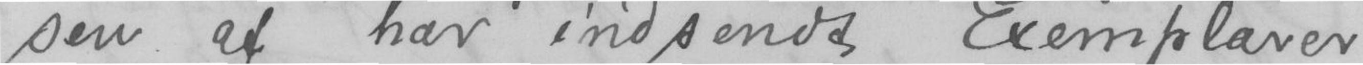sen af har indsendt Exemplarer
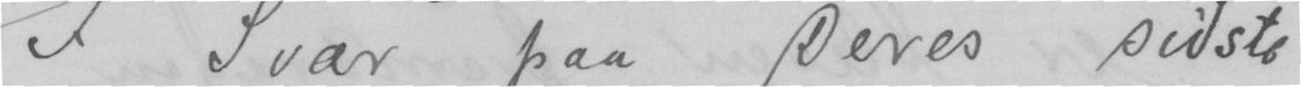I Svar paa Deres sidste
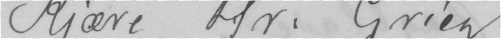Kjære Hr. Grieg!
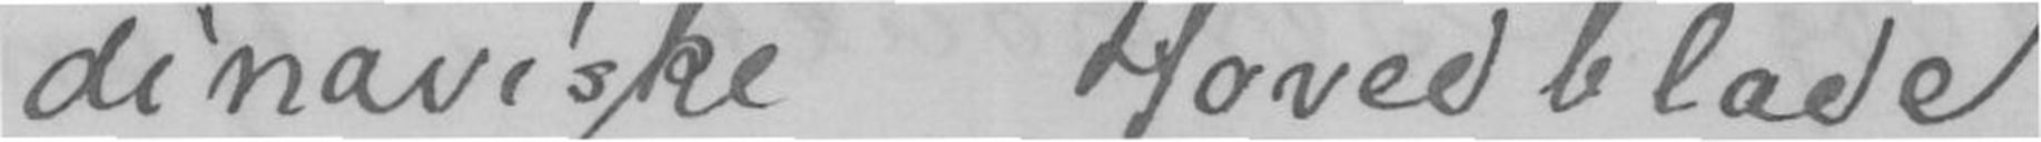dinaviske Hovedblade
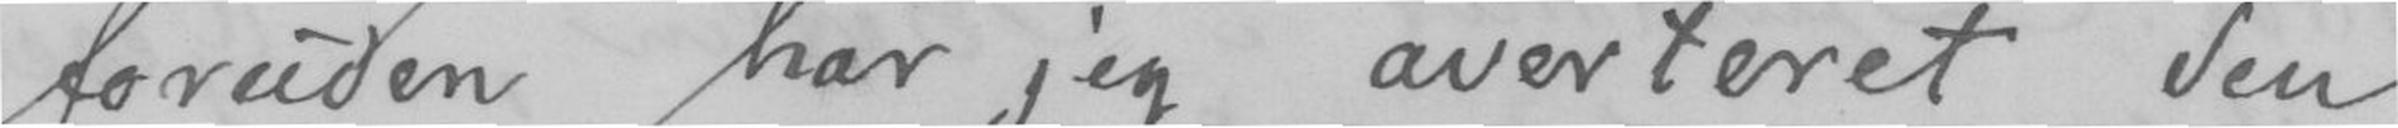foruden har jeg averteret den
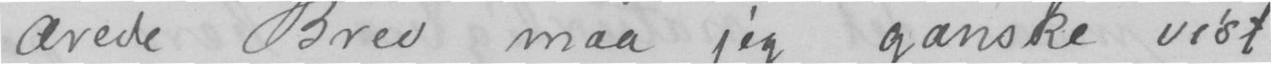Ærede Brev maa jeg ganske vist
